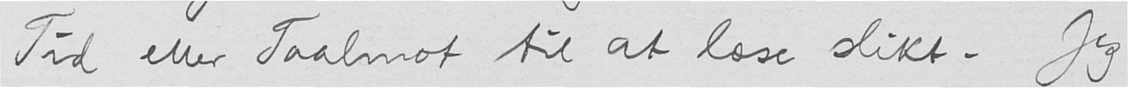Tid eller Taalmot til at læse slikt. Jeg
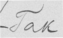Tak
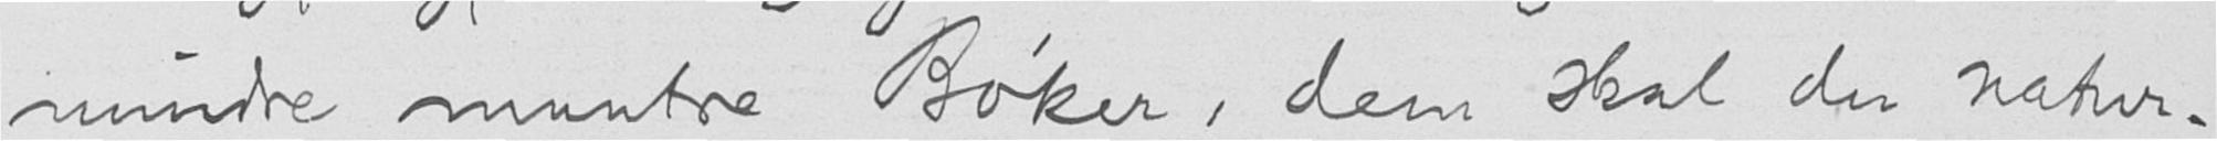mindre muntre Bøker, dem skal du natur-
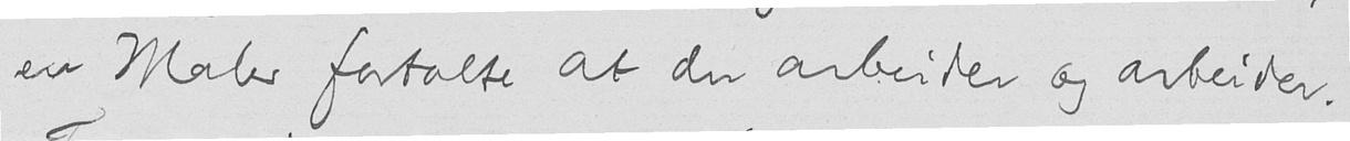en Maler fortalte at du arbeider og arbeider.
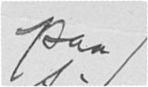paa
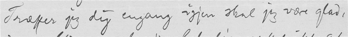Træffer jeg dig engang igjen skal jeg være glad,
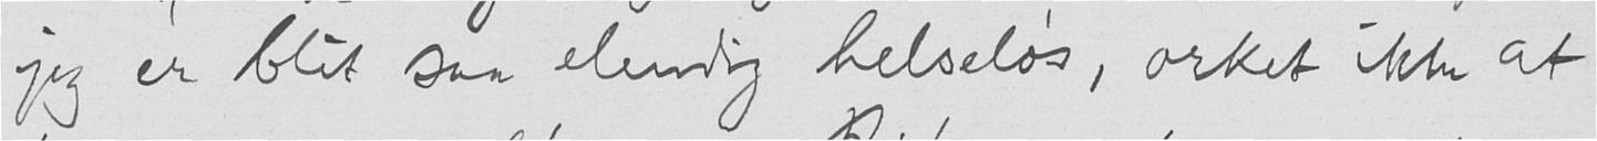jeg er blit saa elendig helseløs, orket ikke at
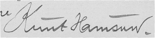Knut Hamsun.
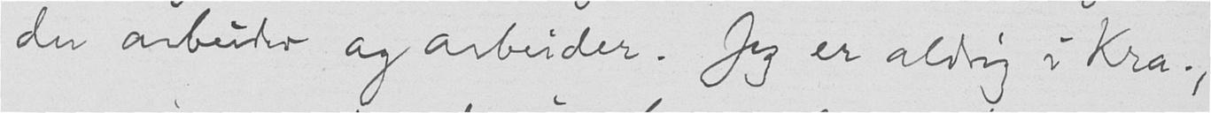du arbeider og arbeider. Jeg er aldrig i Kra.,
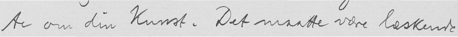te om din Kunst. Det maatte være læskende
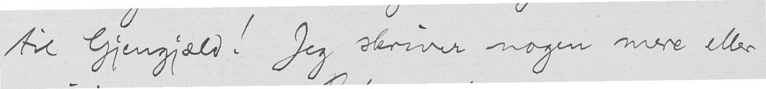til Gjengjæld! Jeg skriver nogen mere eller
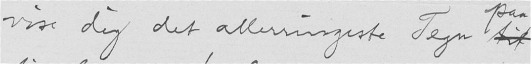vise dig det allerringeste Tegn til
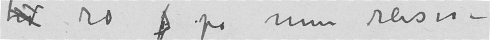tid ropå mine reiser –
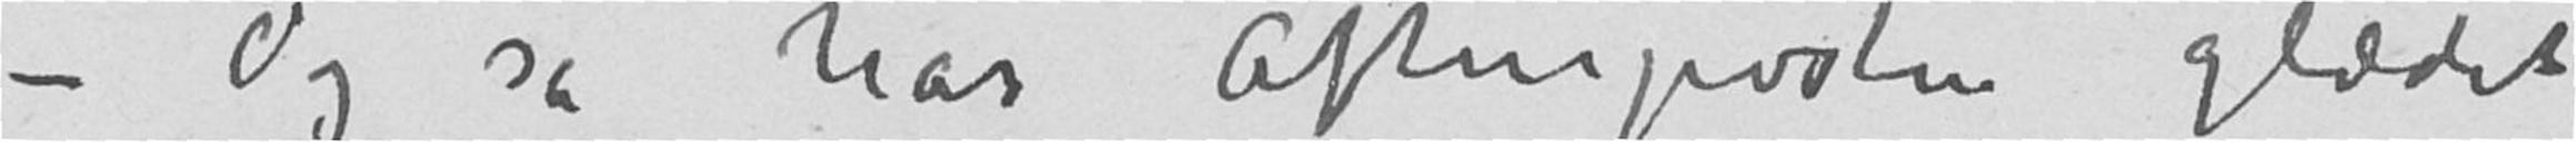– Og så har Aftenposten glædet
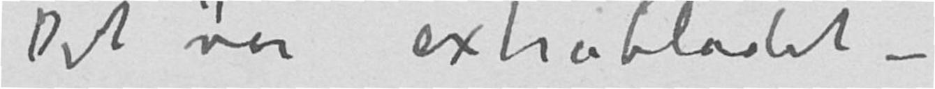Det var extrabladet –
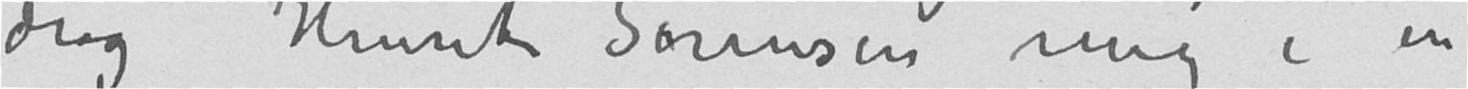drog Henrik Sørensen mig i en
